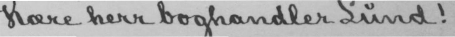Kære herr boghandler Lund!
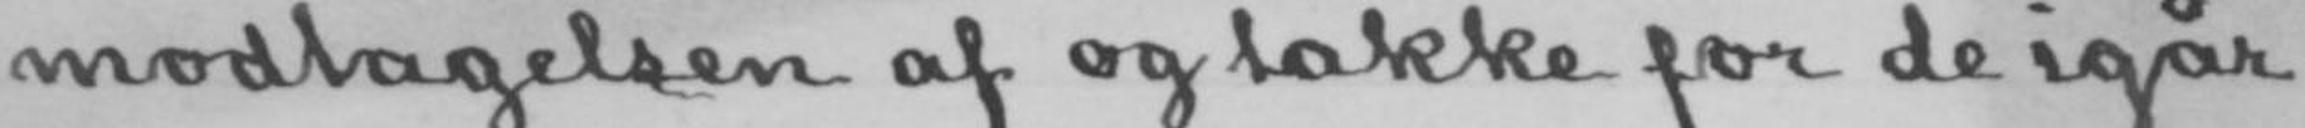modtagelsen af og takke for de igår
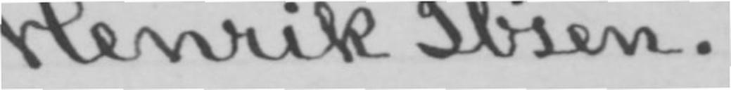Henrik Ibsen.
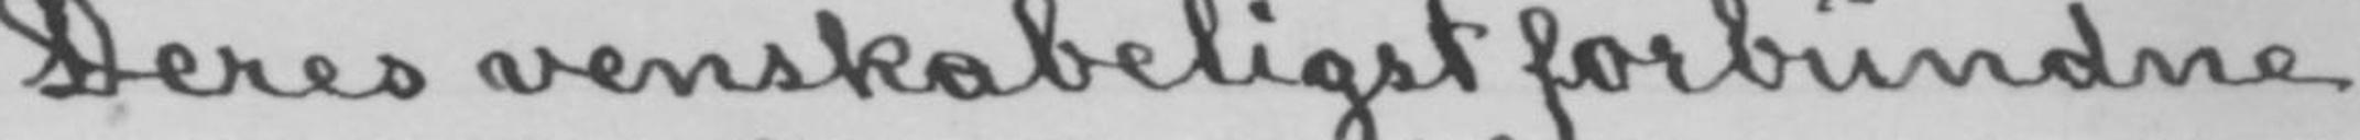Deres venskabeligst forbundne
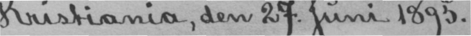Kristiania, den 27. Juni 1895.
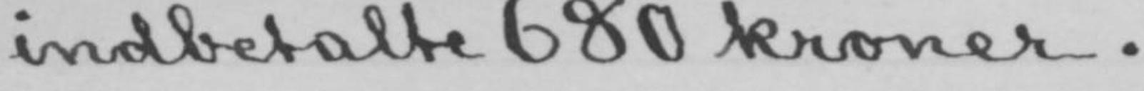indbetalte 680 kroner.
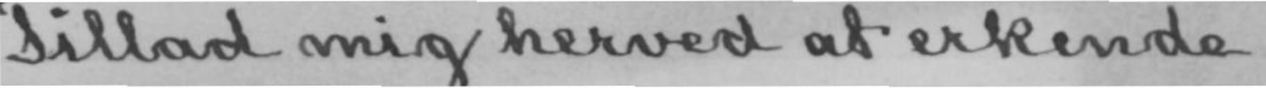Tillad mig herved at erkende
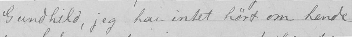Gundhild, jeg har intet hørt om hende
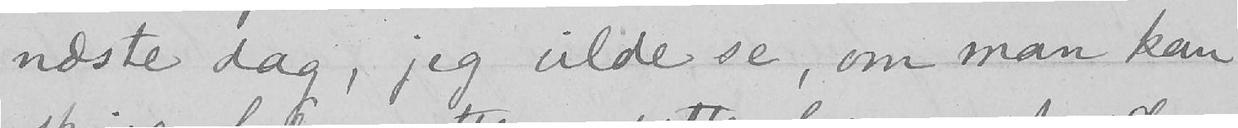næste dag, jeg vilde se, om man kan
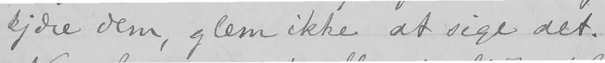kjære dem, glem ikke at sige det.
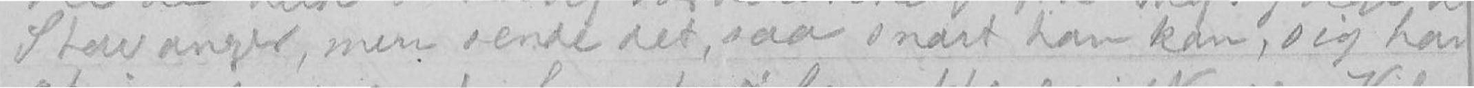Stavanger, men sende det, saa snart han kan, sig han
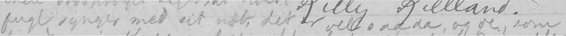fugl synger med sit næb, det er vel saa da, og de, som
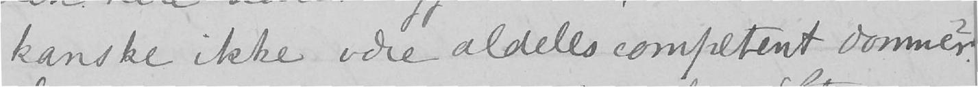kanske ikke være aldeles competent dommer?
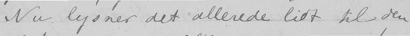Nu lysner det allerede lidt til den
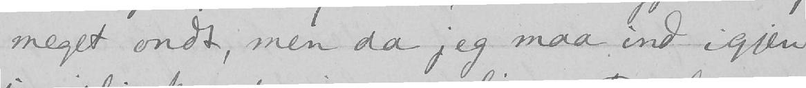meget ondt, men da jeg maa ind igjen
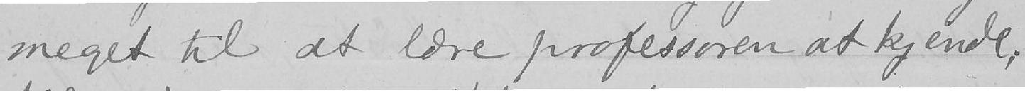meget til at lære professoren at kjende;
